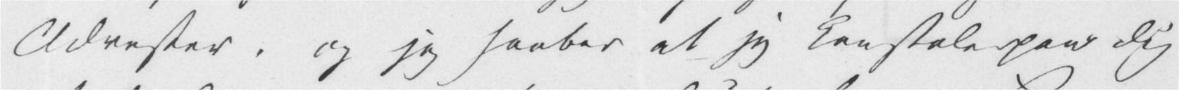Adresser, og jeg haaber at jeg kan stole paa Dig
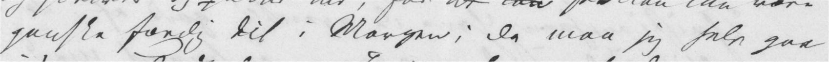ganske færdig til i Morgen; da maa jeg selv gaa
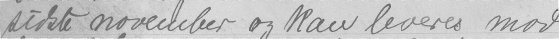sidste november og kan leveres mod
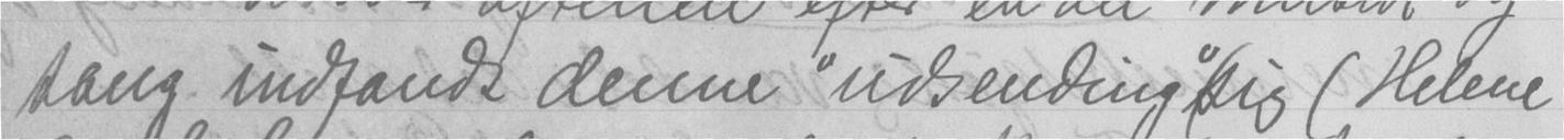sang indfandt denne "udsending" (sig (Helene
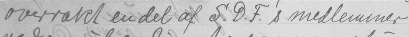overrrakt en del af S.D.F.'s medlemmer
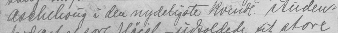Aschehoug i den nydeligste kvindl. studen-
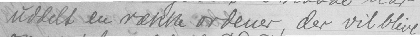uddelt en række ordener, der vil blive
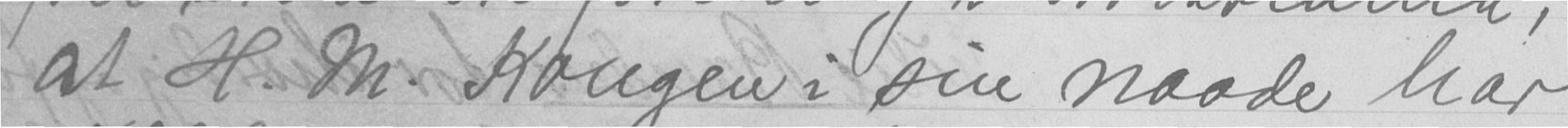at H. M. Kongen i sin naade har
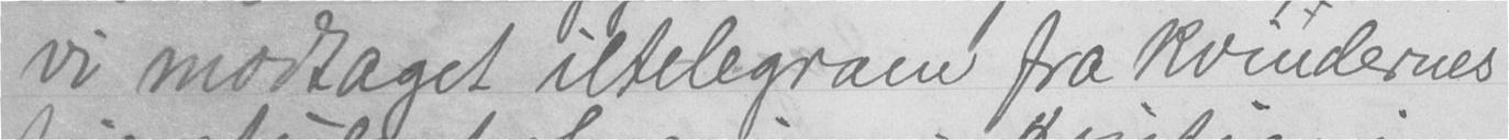vi modtaget iltelegram fra kvindernes
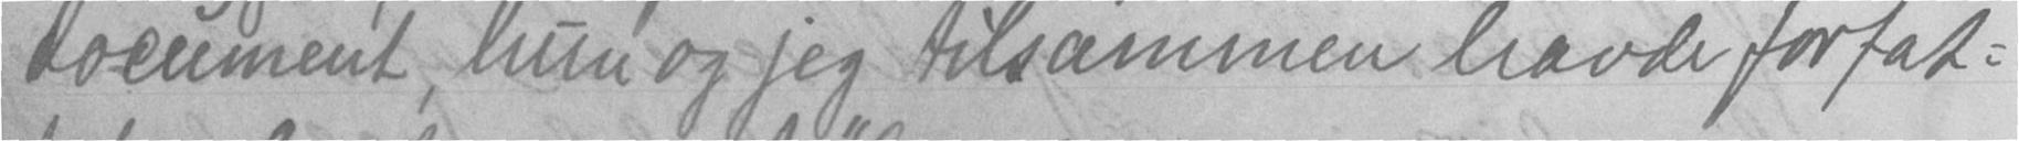document, hun og jeg tilsammen havde forfat-
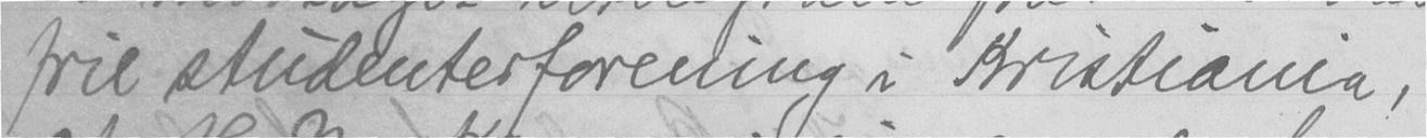frie studenterforening i Kristiana,
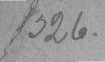326.
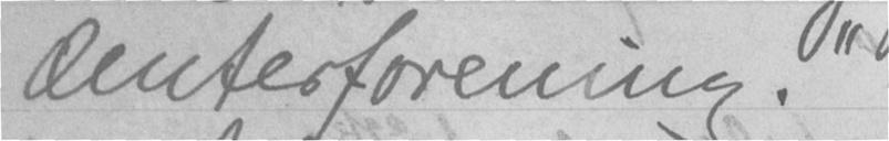denterforening."
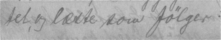tet, og læste som følger:
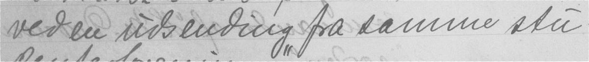ved en udsending fra samme stu-
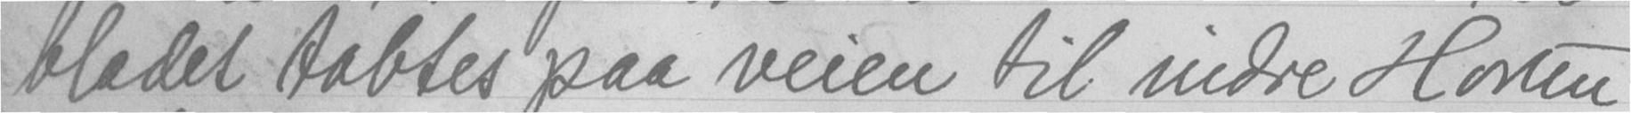bladet tabtes paa veien til indre Horten
